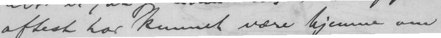oftest har kunnet være hjemme om
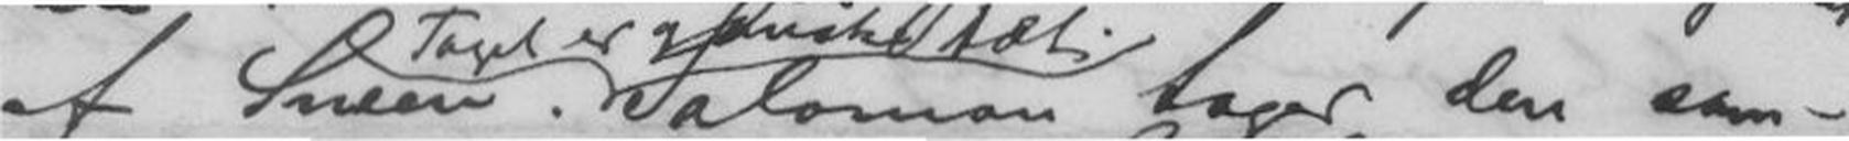af Sneen. Salomon tager den sam-
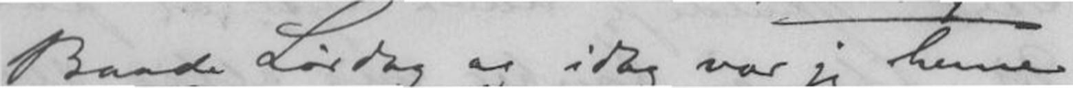Baade Lørdag og idag var jeg heme
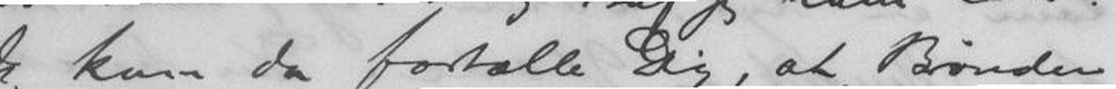Jeg kan da fortælle Dig, at Brønden
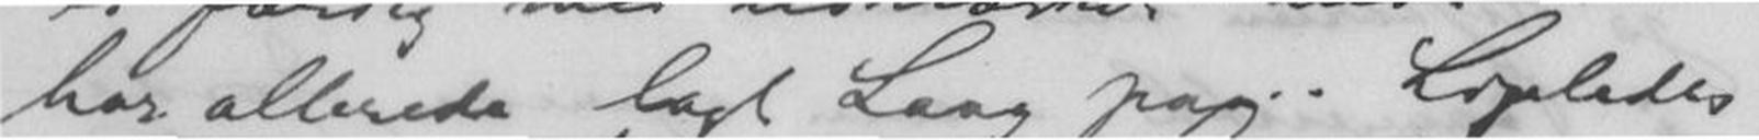har allerede lagt Laag paa. Ligeledes
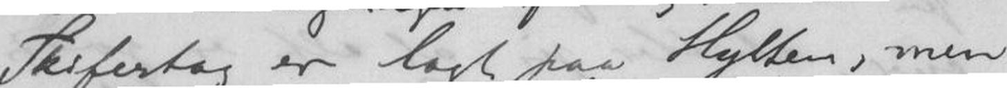Skifertag er lagt paa Hytten, men
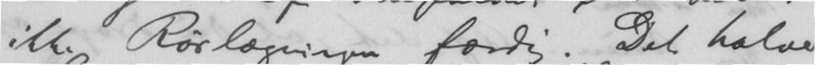ikke Rørlægningen færdig. Det halve
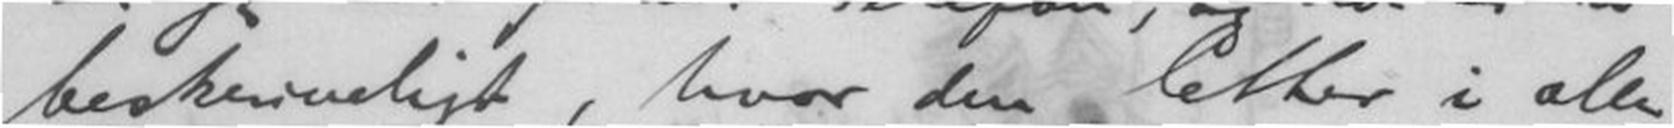beskriveligt, hvor den letter i alle
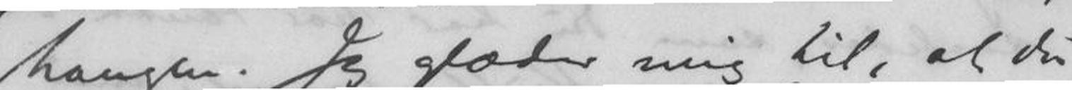haugen. Jeg glæder mig til, at du
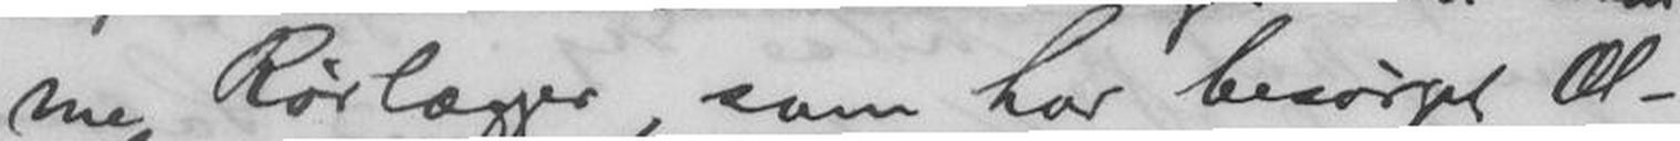me Rørlægger, som har besørget Al-
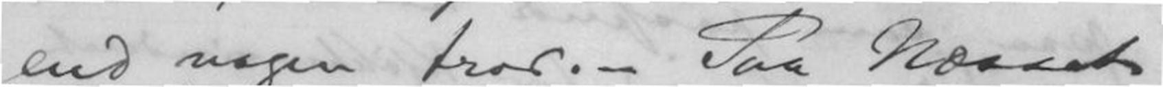end nogen tror. - Paa Næsset
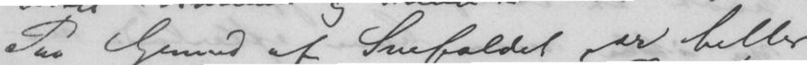Paa Grund af Snefaldet er heller
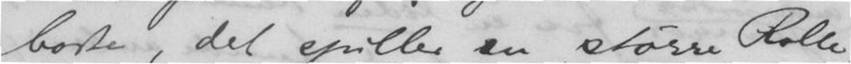borte, det spiller en større Rolle,
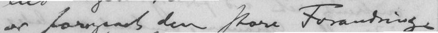er foregaaet den store Forandring
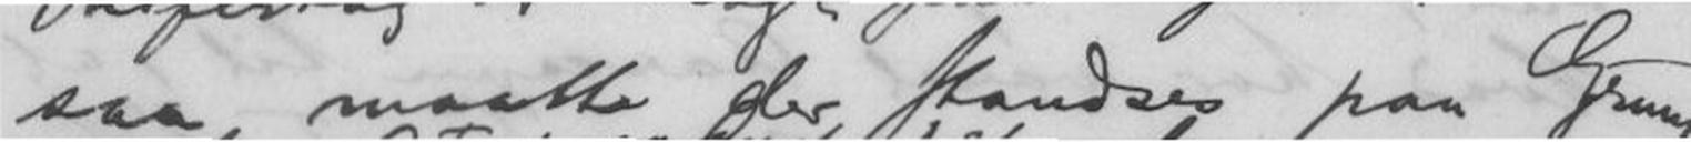saa maatte der standses paa Grund
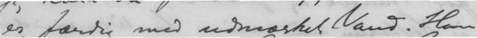er færdig med udmærket Vand. Han
